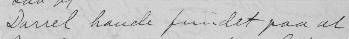Darrel havde fundet paa at
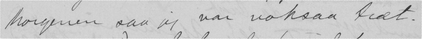Morgenen saa jeg var noksaa træt.
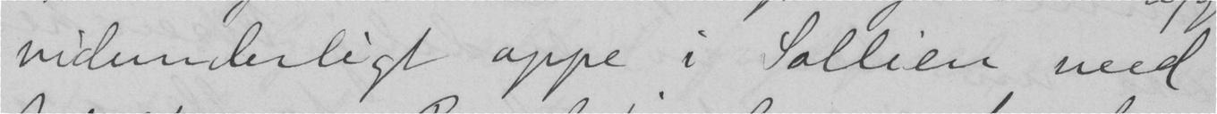vidunderligt oppe i Sollien med
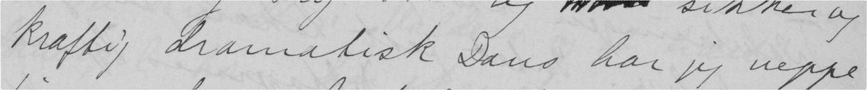kraftig dramatisk Dans har jeg neppe
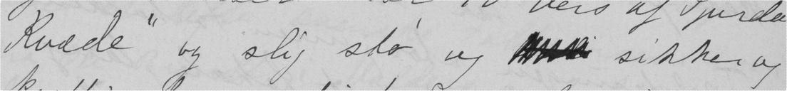Kvæde" og slig stø og  sikker og
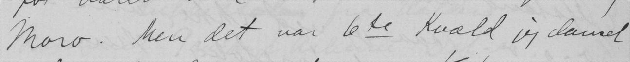Moro. Men det var 6te Kvæld jeg danset
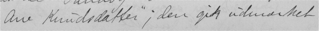Ane Knudsdatter"; den gik udmærket
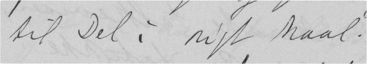til Del i rigt Maal.
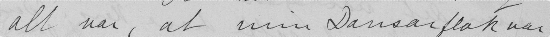alt var, at min Dansarflok var
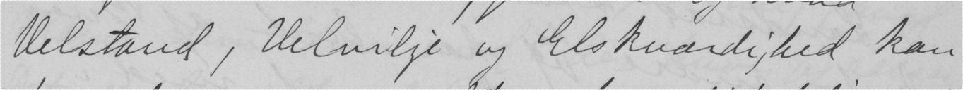Velstand, Velvilje og Elskværdighed kan
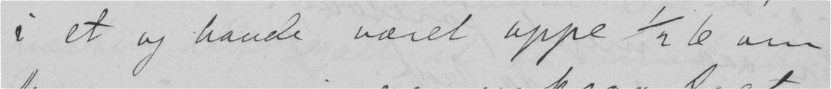i et og havde været oppe 1/2 6 om
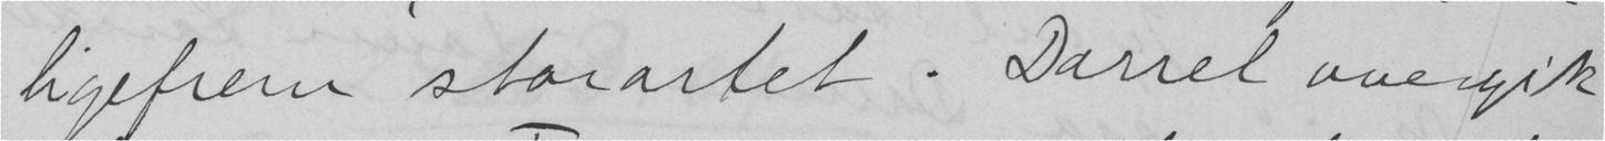ligefrem storartet. Darrel overgik
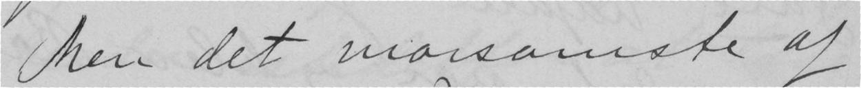Men det morsomste af
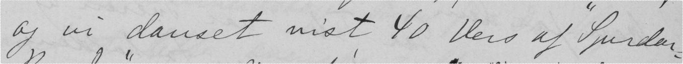og vi danset vist 40 Vers af "Sjurdar-
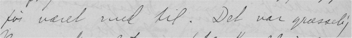før været med til. Det var græsselig
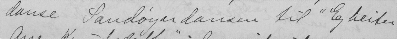danse Sandøyardansen til "Eg heiter
